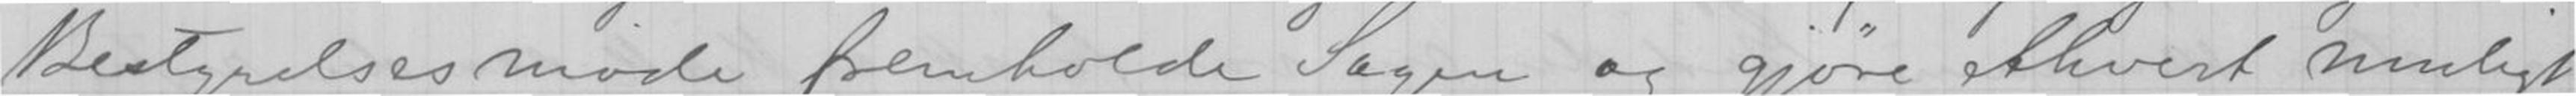Bestyrelsesmøde fremholde Sagen og gjøre ethvert muligt
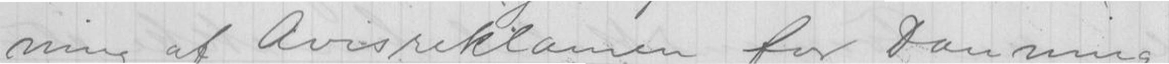ning af Avisreklamen for Danning
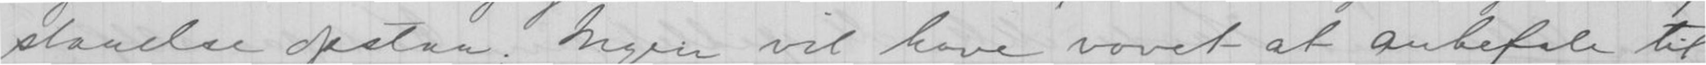staaelse opstaa; Ingen vil have vovet at anbefale til
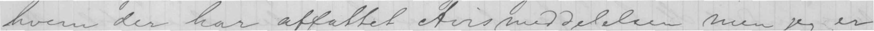hvem der har affattet Avismeddelelsen, men jeg er
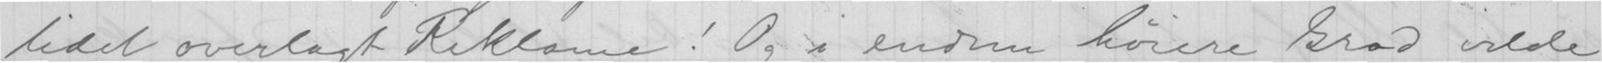lidet overlagt Reklame! Og i endnu høiere Grad vilde
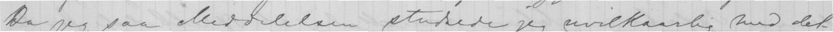Da jeg saa Meddelelsen studsede jeg uvilkaarlig med det
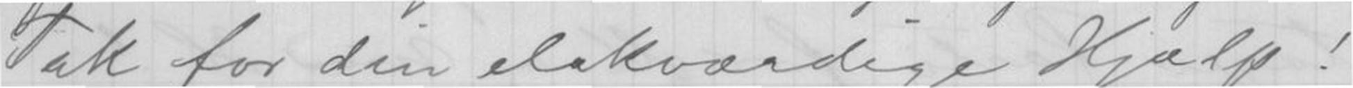Tak for din elskværdige Hjælp!
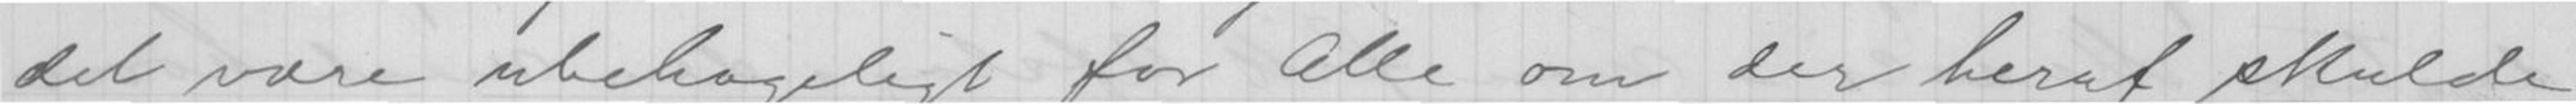det være ubehageligt for Alle om der heraf skulde
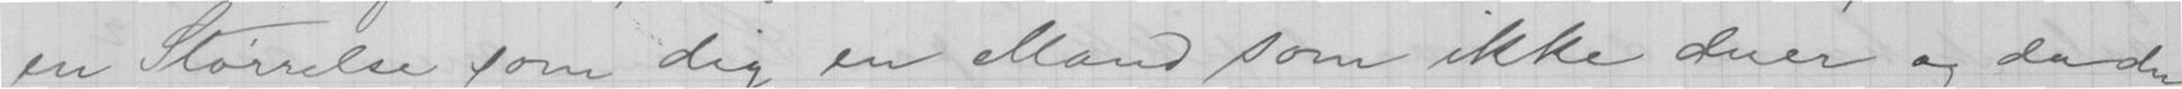en Størrelse som dig en Mand som ikke duer og da du
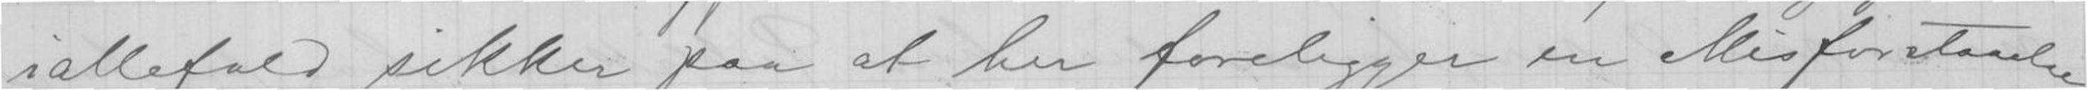iallefald sikker paa at her foreligger en Misforstaaelse
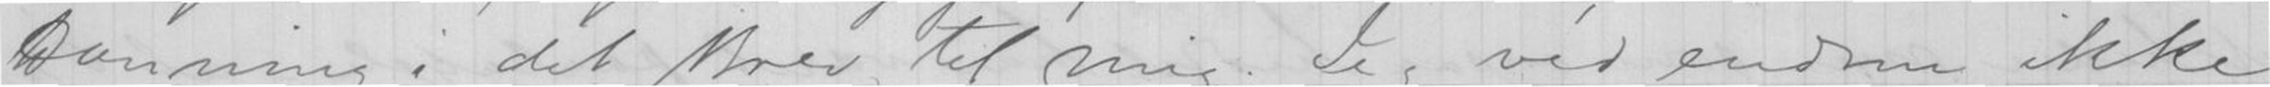Danning i det Brev til mig. Jeg véd endnu ikke
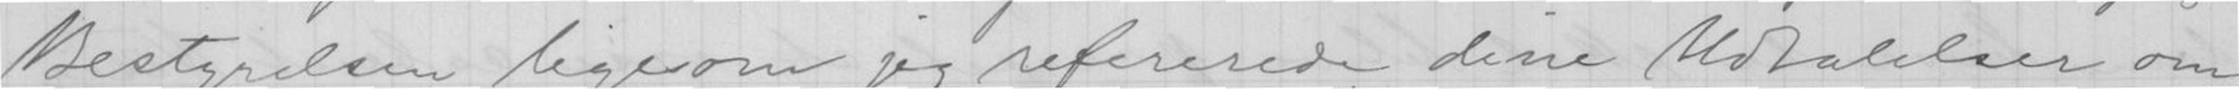Bestyrelsen ligesom jeg refererede dine Udtalelser om
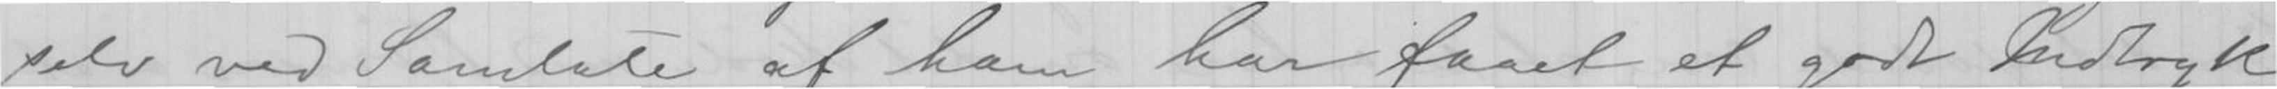selv ved Samtale af ham har faaet et godt Indtryk
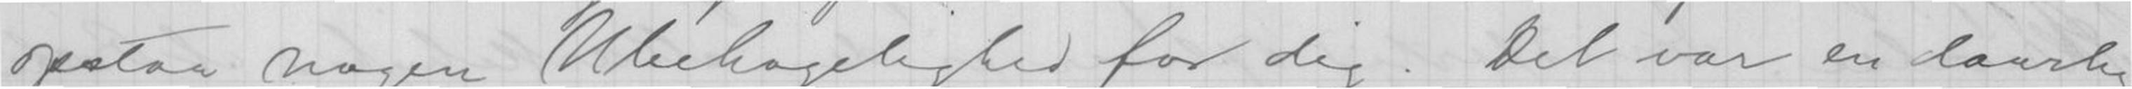opstaa nogen Ubehagelighed for dig. Det var en daarlig
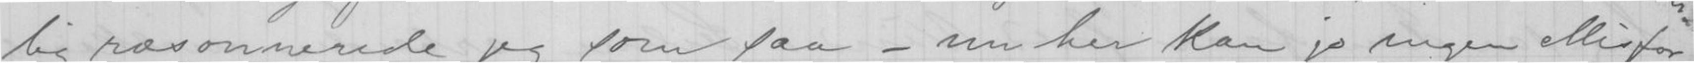lig ræsonnerede jeg som saa - nu, her kan jo ingen Misfor-
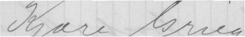Kjære Grieg!
